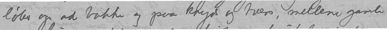løber op ad bakke og paa kryds og tvers, mellem gamle
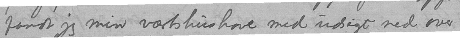fandt jeg min værtshushave med udsigt ned over
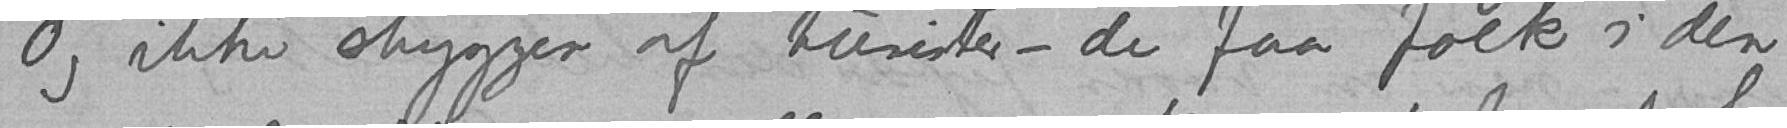Og ikke skyggen af turister - de faa folk i den
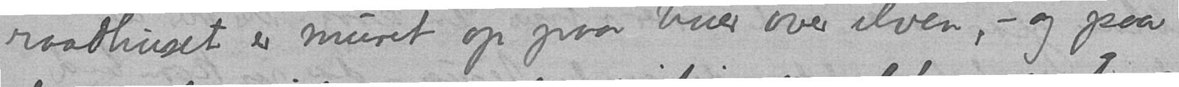raadhuset er muret op paa buer over elven, - og paa
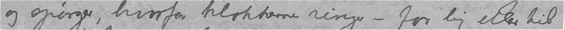og spørger, hvorfor klokkerne ringer - for lig eller til
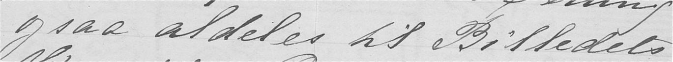ogsaa aldeles til Billedets
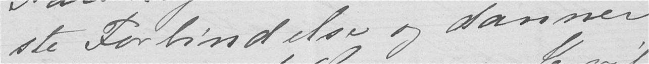ste Forbindelse og danner
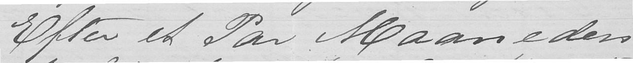Efter et Par Maaneders
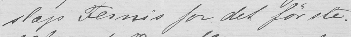slags Fernis for det første.
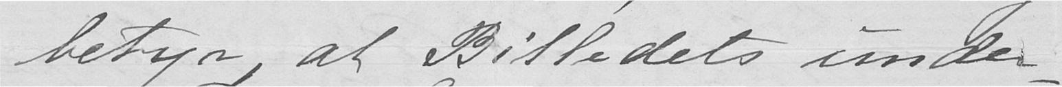betyr at Billedets under-
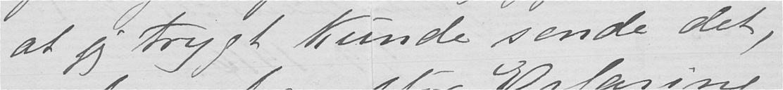at jeg trygt kunde sende det,
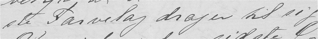ste Farvelag drager til sig
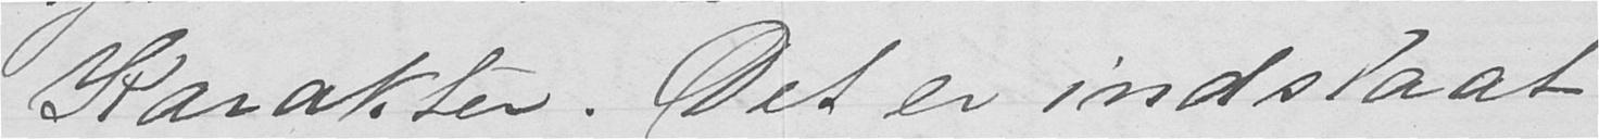Karakter. Det er indslaat
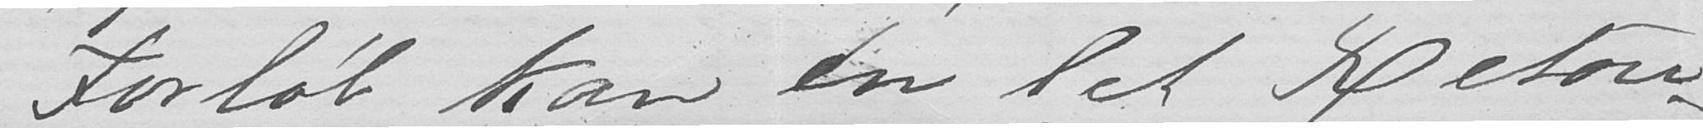Forløb kan en let Retou-
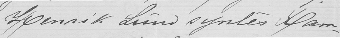Henrik Lund syntes Ram-
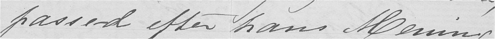passed efter hans Mening
